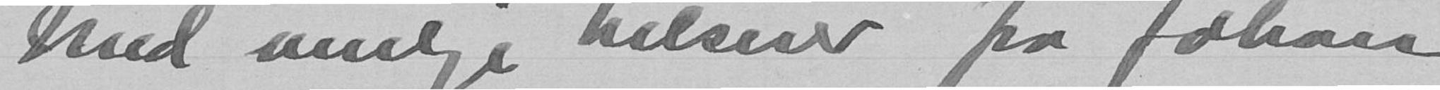Med venlige hilsner fra Johan
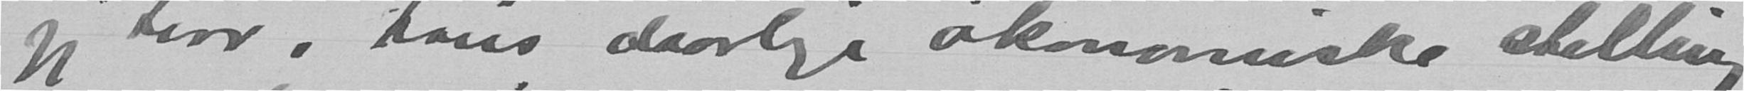jeg tror, hans daarlige økonomiske stilling
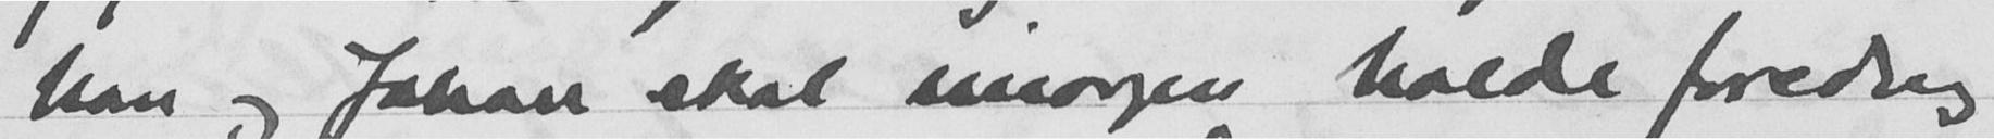han og Johan skal imorgen holde foredrag
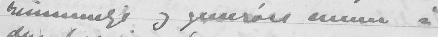rummerlige og generøse menn i
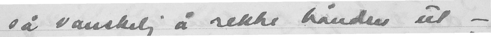så vanskelig å rekke hånden ut -
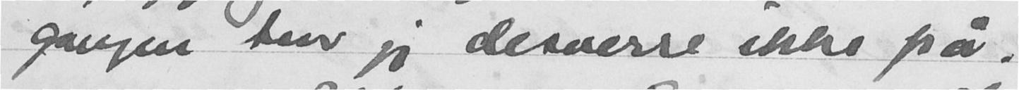gangen tror jeg dessverre ikke på.
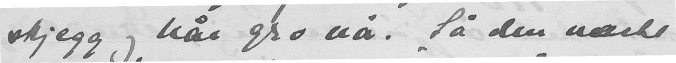skjegg og hår gro nå. Så den næste
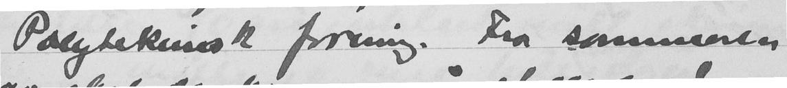Polyteknisk forening. Fra sommeren
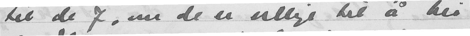til de 7, om de er villige til a bli
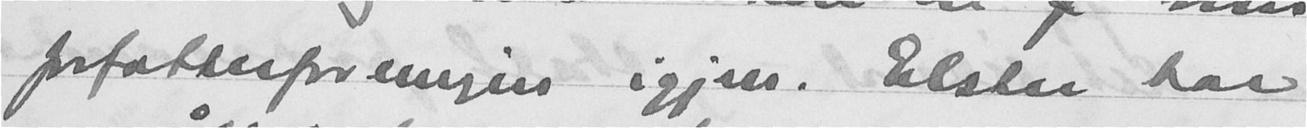forfatterforeningen igjen. Elster har
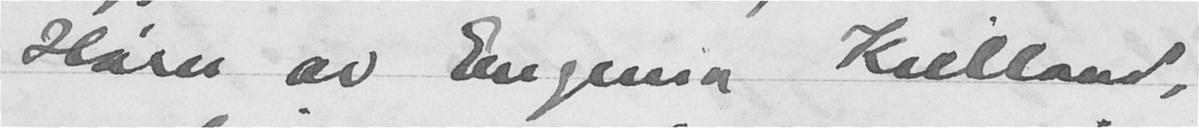Hører av Eugenia Kielland,
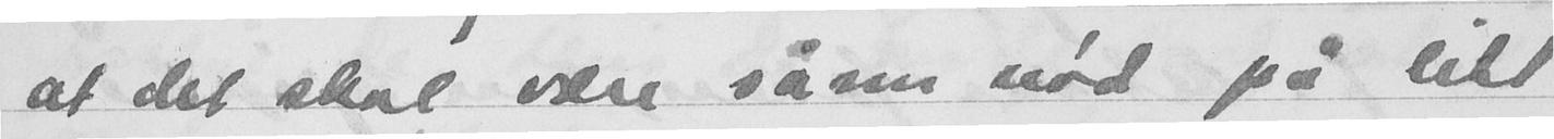at det skal være så mye nød på litt
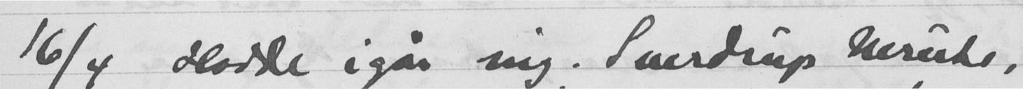16/4 Hadde igår mig. Sverdrup herute,
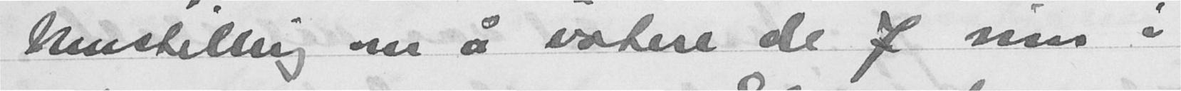innstilling om å votere de 7 inn i
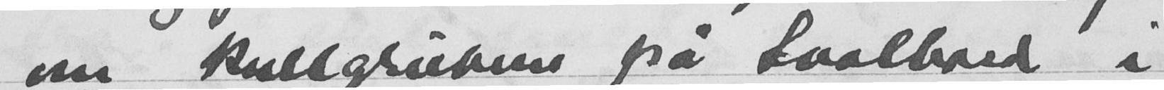om Kullgruven på Svalbard i
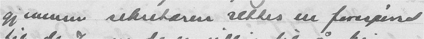gjennom sekretæren rettes en forespørsel
